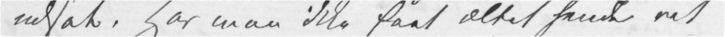udsat. Har man ikke fået æltet hende ret
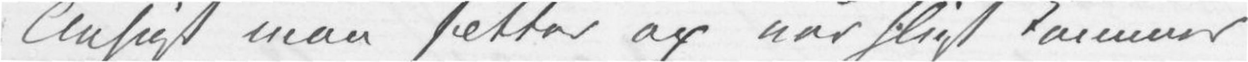Ansigt man sætter op når sligt kommer
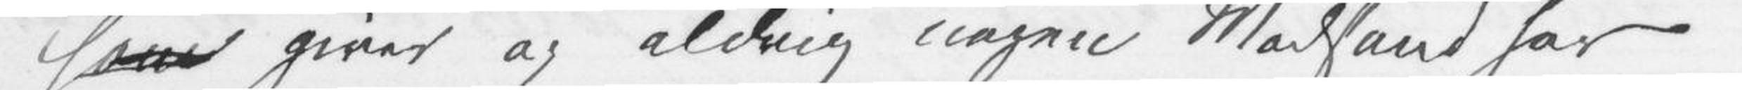som giver og aldrig nogen Modstand har
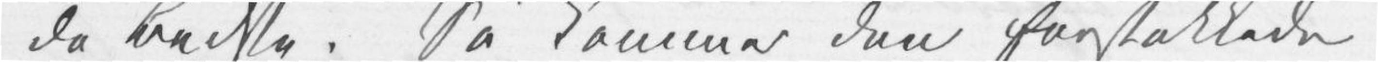de Bedste. Så kommer den forstokkede
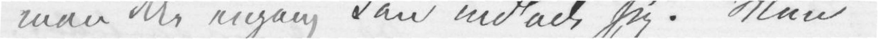man ikke engang kan indlade sig. Man
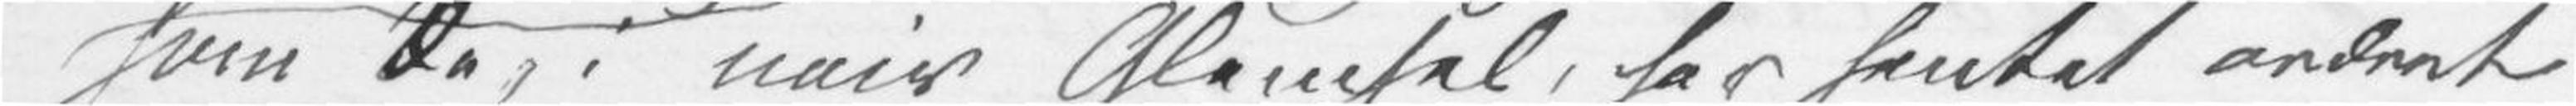som de, i naiv Glemsel, har hentet ordret
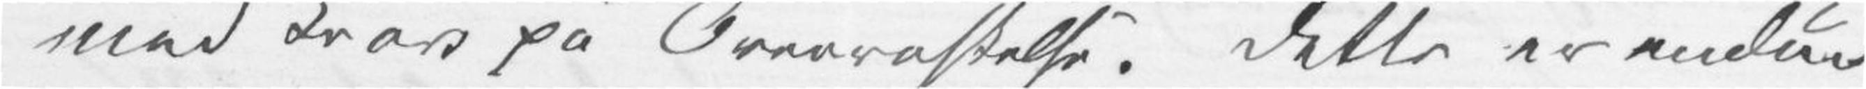med Krav på Overraskelse. Dette er endnu
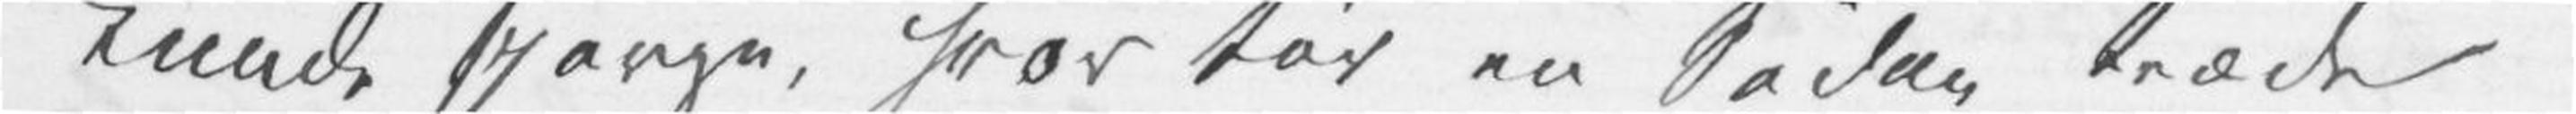kunde spørge, hvor tør en Sådan træde
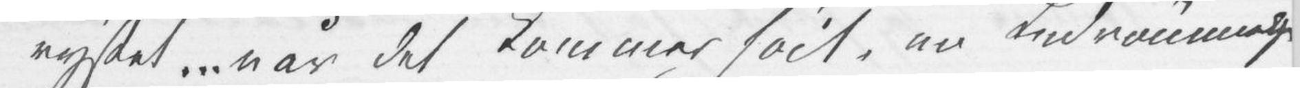rystet ... når det kommer høit, en Indrømmelse
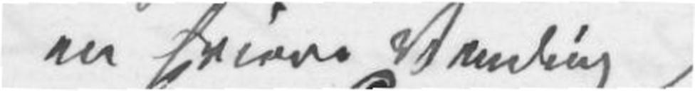en friere Vending
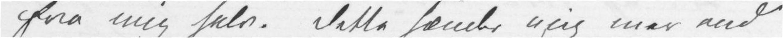fra mig selv. Dette hænder mig mer end
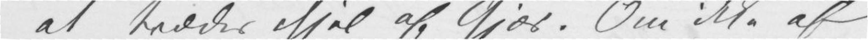at trædes ihjel af Gjæs. Om ikke af
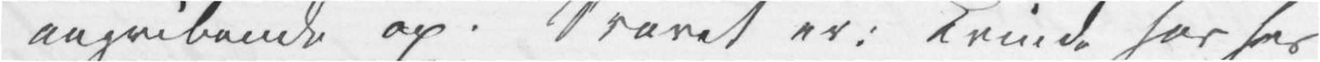angribende op? Svaret er: Kvinde har her
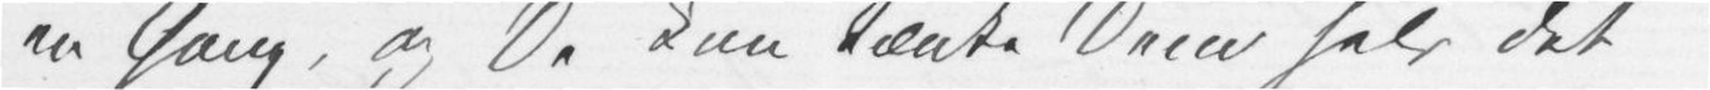en Gang, og De kan tænke Dem selv det
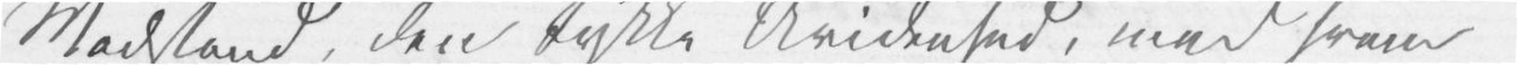Modstand, den tykke Uvidenhed, med hvem
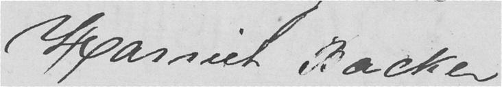Harriet Backer
